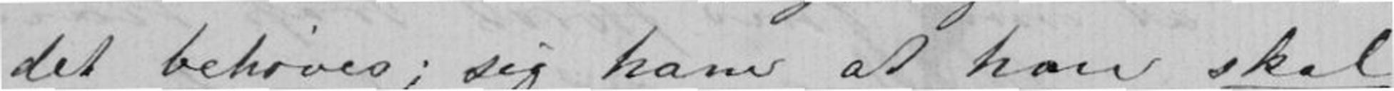det behøves; sig ham at han skal
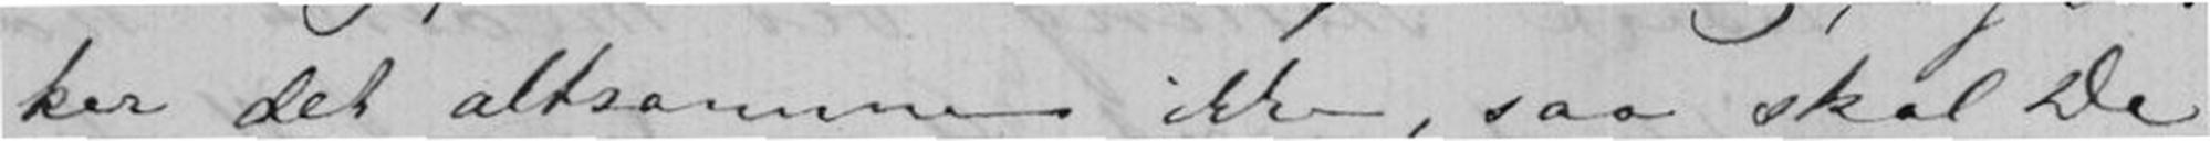ker det altsammen ikke, saa skal De
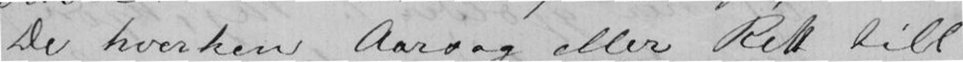De hverken Aarsag eller Rett till
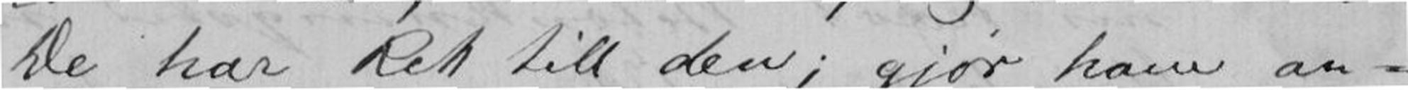De har Rett till den; gjør ham an-
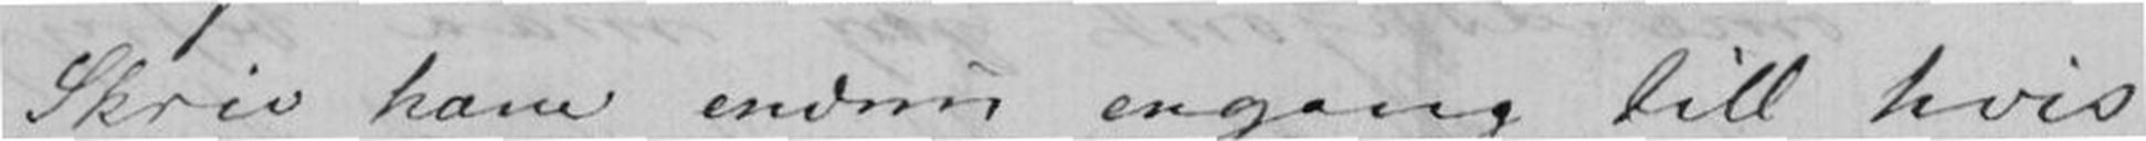Skriv ham endnu engang till hvis
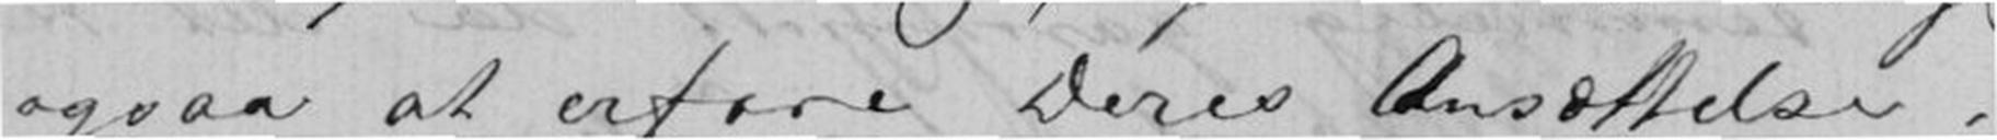ogsaa at erfare Deres Ansættelse.
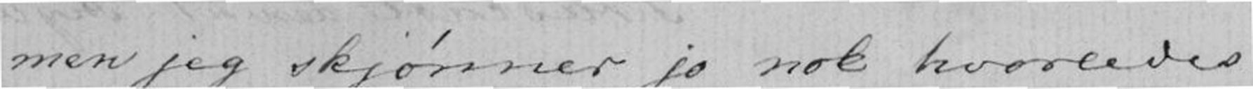men jeg skjønner jo nok hvorledes
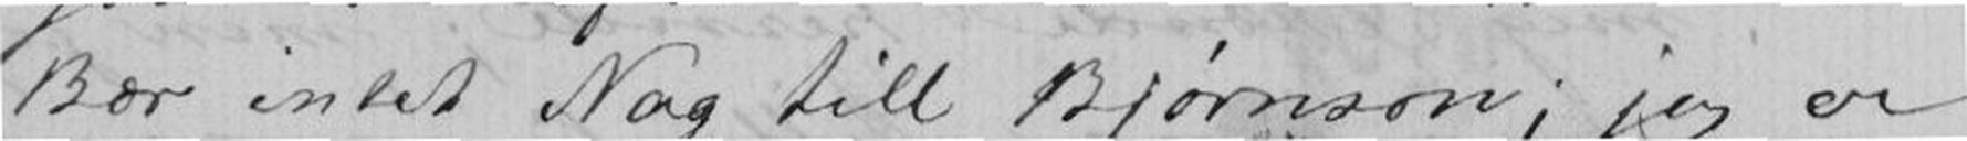Bær intet Nag till Bjørnson; jeg er
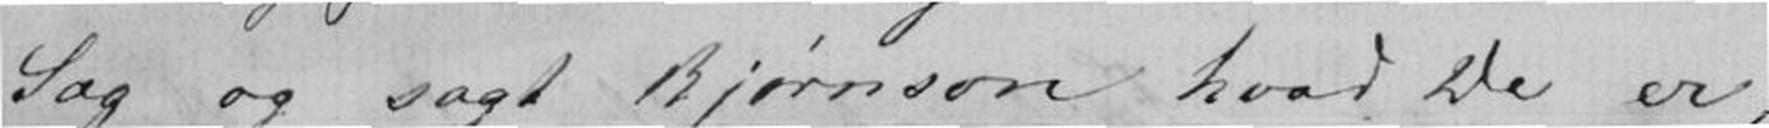Sag og sagt Bjørnson hvad De er
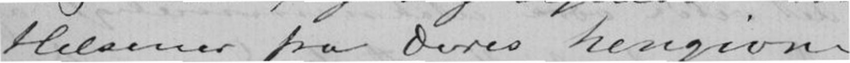Hilsener fra Deres hengivne
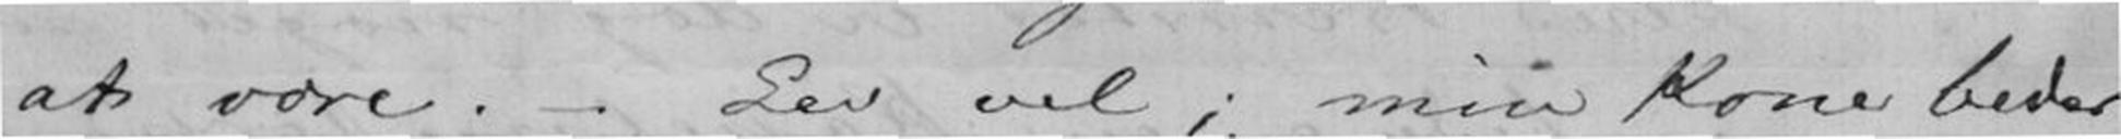at være. - Lev vel; min Kone beder
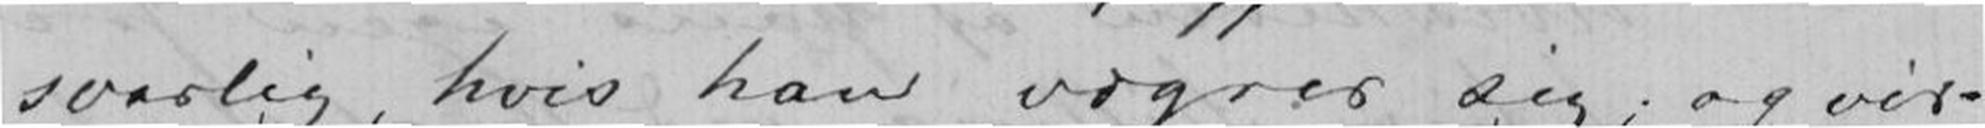svarlig, hvis han vægrer sig; og vir-
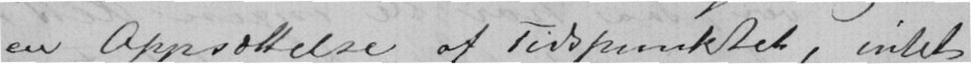en Oppsættelse af Tidspunktet, intet
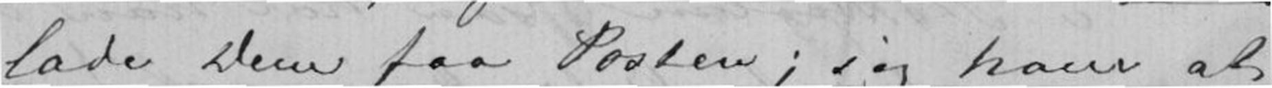lade Dem faa Posten; sig ham at
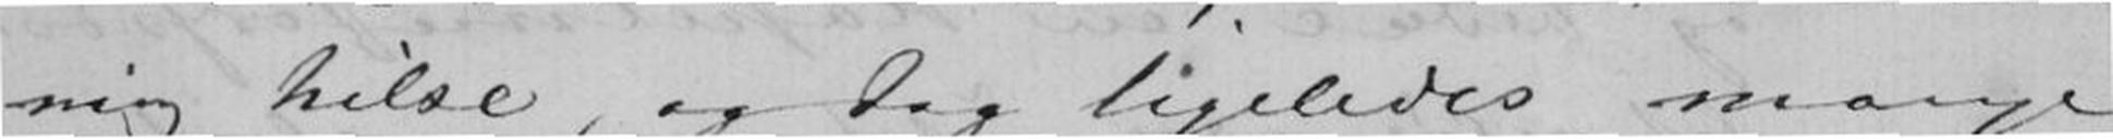mig hilse, og tag ligeledes mange
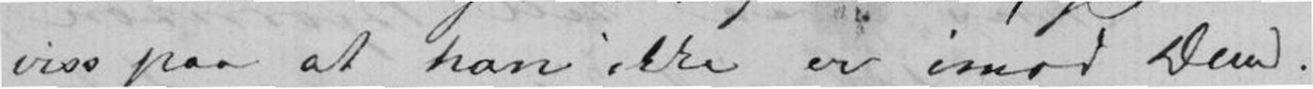viss paa at han ikke er imod Dem.
